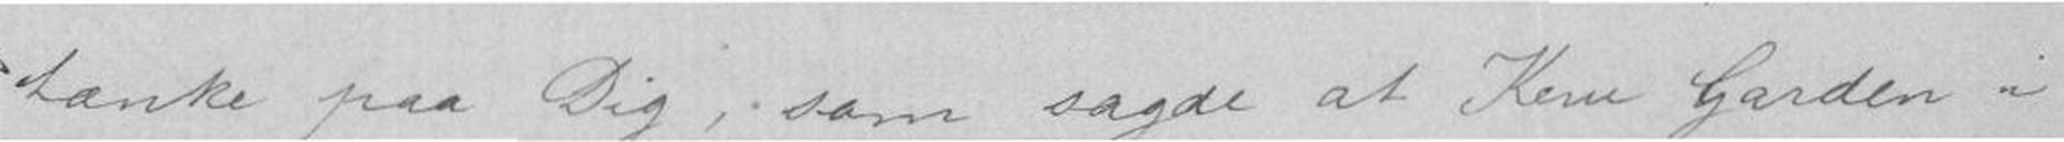tænke paa Dig, som sagde at Kenc Garden i
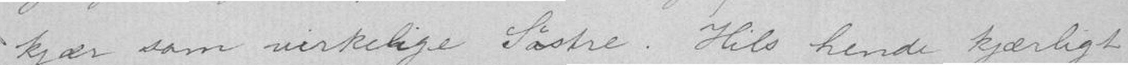kjær som virkelige Søstre. Hils hende kjærligt
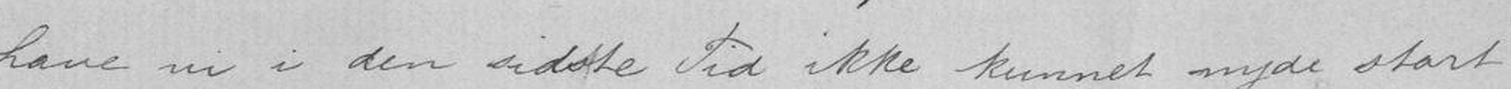have vi i den sidste Tid ikke kunnet nyde stort
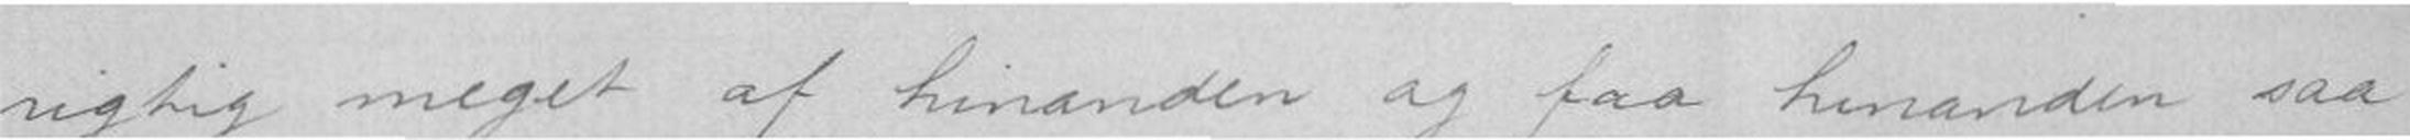rigtig meget af hinanden og faa hinanden saa
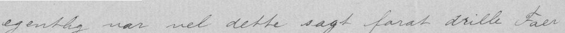egentlig var dette sagt forat drille Faer
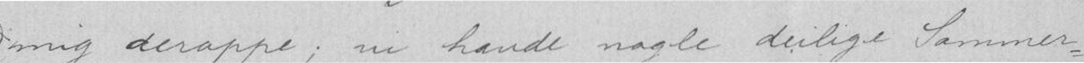mig deroppe; vi havde nogle deilige Sommer-
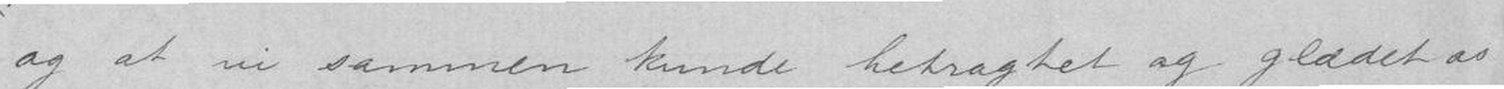og at vil sammen kunde betragtet og glædet os
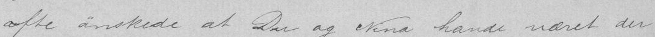ofte ønskede at Du og Nina havde været der
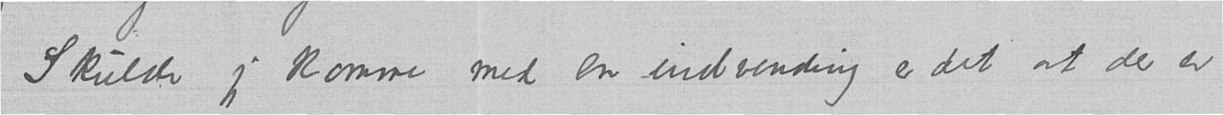Skulde jeg komme med en indvending er det at der er
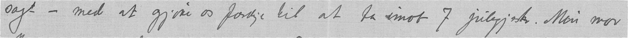sagt – med at gjøre os færdige til at ta imot 7 julegjester. Min mor
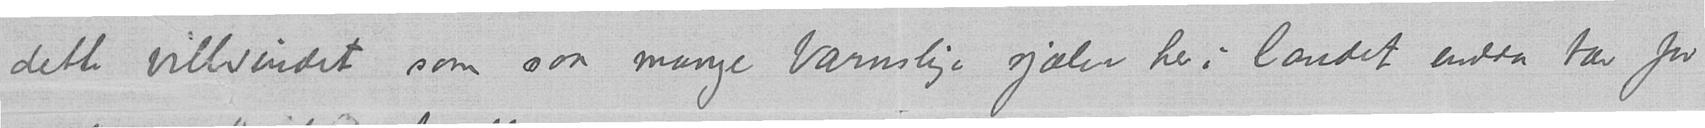dette villsindet som saa mange barnslige sjæler her i landet endda tar for
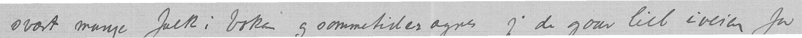svært mange folk i boken og sommetider synes jeg de gaar litt iveien for
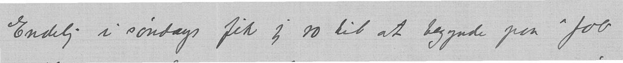Endelig i søndags fik jeg ro til at begynde paa "Job
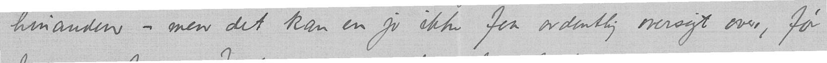hinanden – men det kan en jo ikke faa ordentlig oversigt over, før
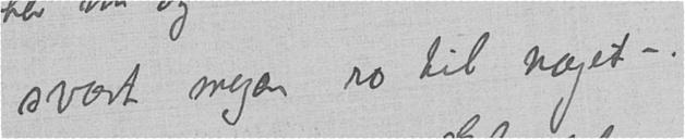svært megen ro til noget –.
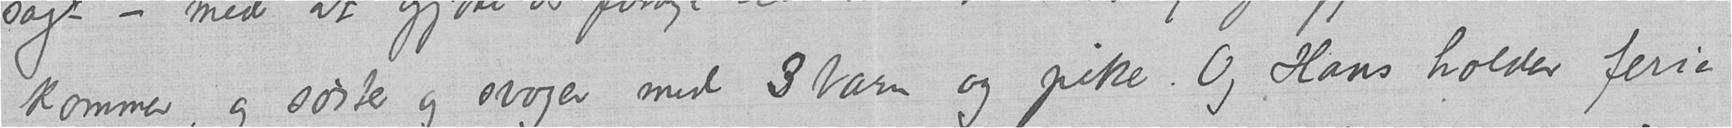kommer, og søster og svoger med 3 barn og pike. Og Hans holder ferie
